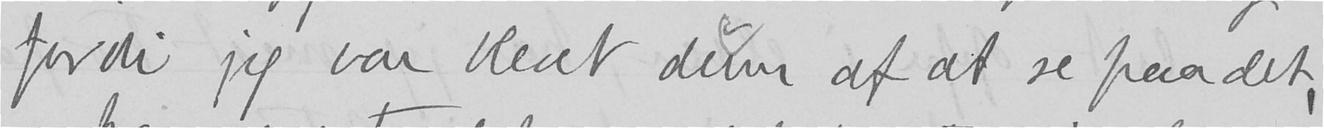fordi jeg var blevet dum af at se paa det,
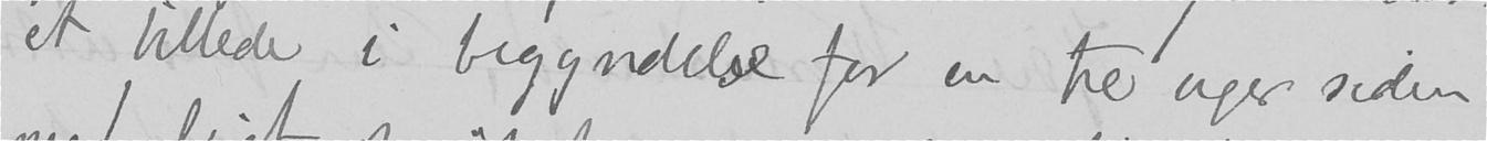et billede i begyndelse for en tre uger siden
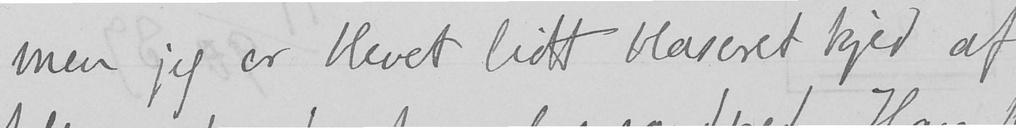men jeg er blevet lidt blaseret kjed af
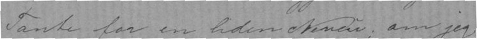Tante for en liden Neveu; om jeg
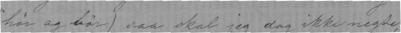hør og bør) saa skal jeg ikke negte,
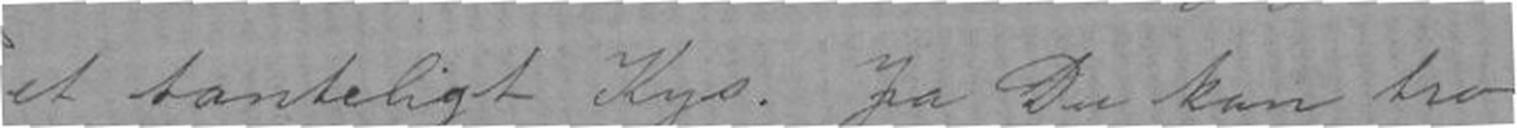et tantelig Kys. Ja Du kan tro,
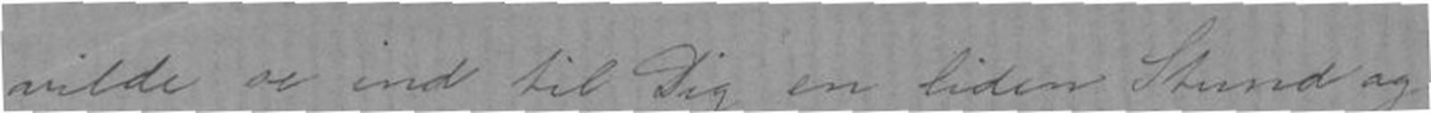vilde se ind til Dig en liden Stund og
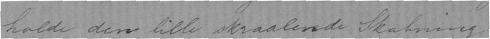holde den lille skraalende Skabning
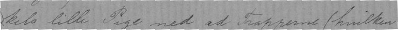kels lille Pige ned ad Trapperne (hvilken
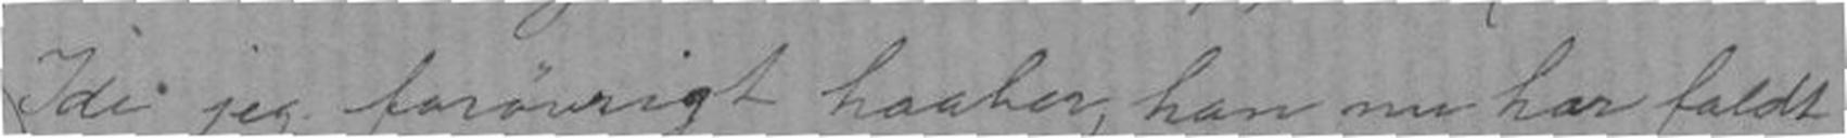Ide jeg forøvrigt haaber, han nu har faldt
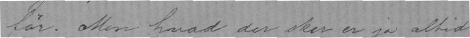før. Men hvad der sker er jo altid
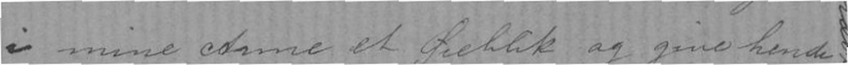i mine Arme et Øieblik og give hende
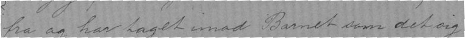fra og har taget imod Barnet som det sig
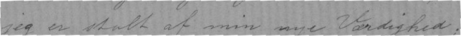jeg er stolt at min nye Værdighed;
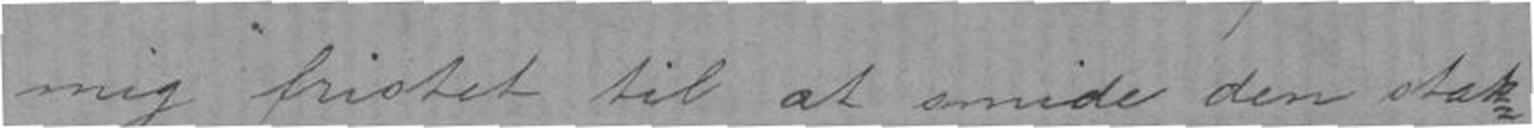mig fristet til at smide den stak-
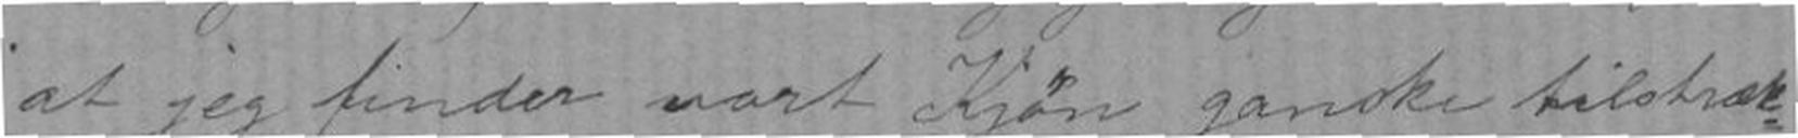at jeg finder vort Kjøn ganske tilstræk-
